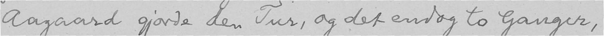Aagaard gjorde den Tur, og det endog to Ganger,
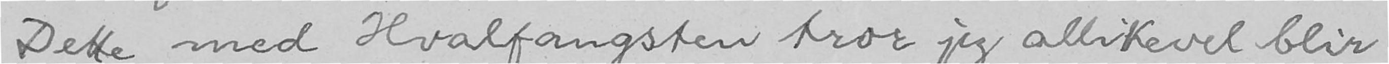Dette med Hvalfangsten tror jeg allikevel blir
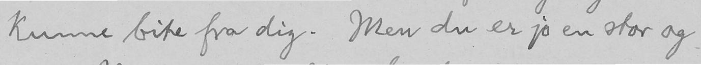kunne bite fra dig. Men du er jo en stor og
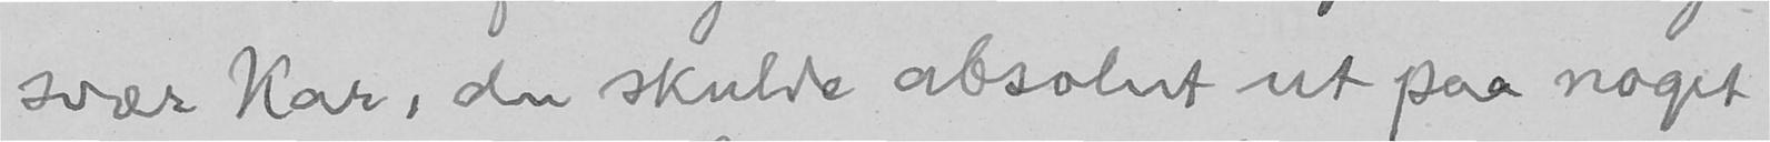svær Kar, du skulde absolut ut paa noget
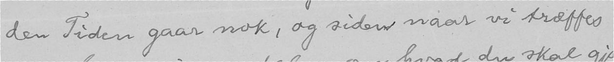den Tiden gaar nok, og siden naar vi træffes
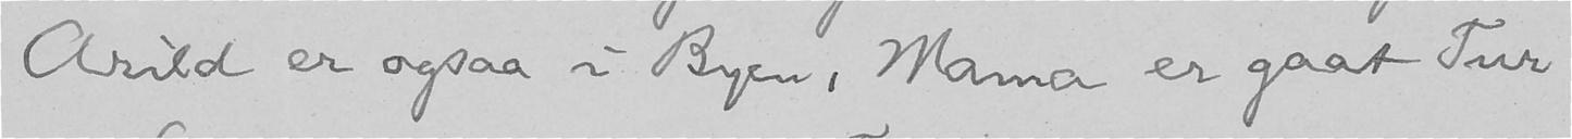Arild er ogsaa i Byen, Mama er gaat Tur
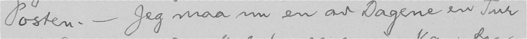Posten. - Jeg maa nu en av Dagene en Tur
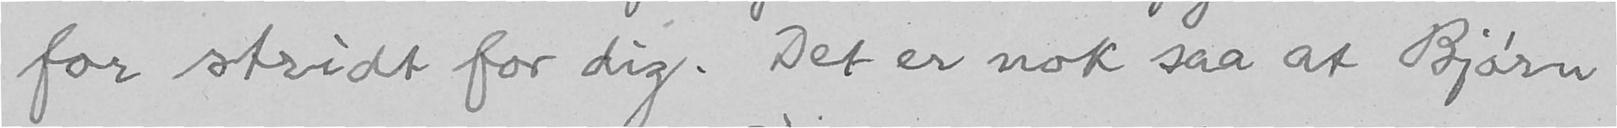for stridt for dig. Det er nok saa at Bjørn
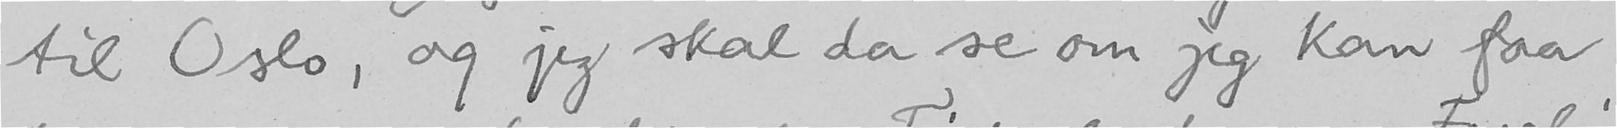til Oslo, og jeg skal da se om jeg kan faa
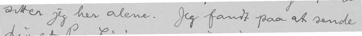sitter jeg her alene. Jeg fandt paa at sende
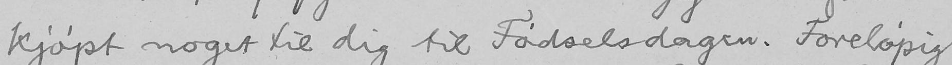kjøpt noget til dig til Fødselsdagen. Foreløpig
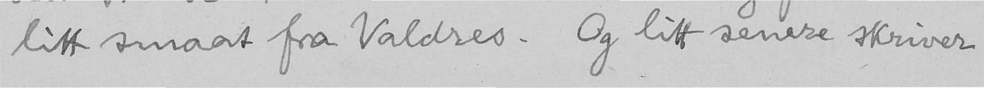litt smaat fra Valdres. Og litt senere skriver
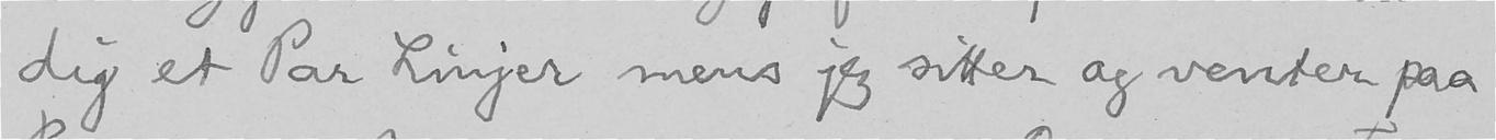dig et Par Linjer mens jeg sitter og venter paa
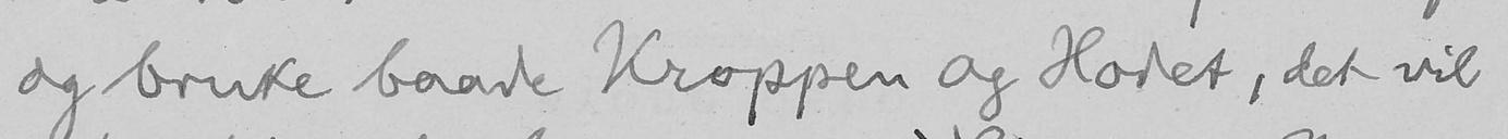og bruke baade Kroppen og Hodet, det vil
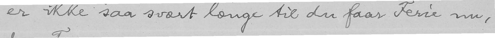er ikke saa svært længe til du faar Ferie nu,
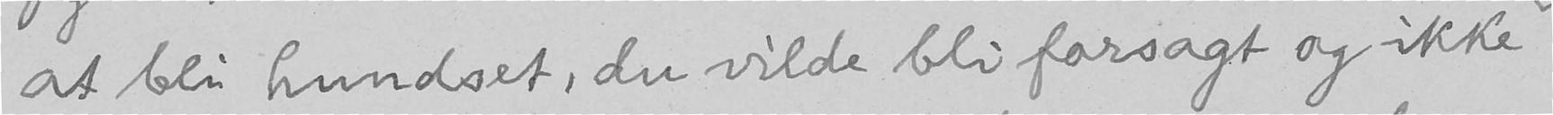at bli hundset, du vilde bli forsagt og ikke
